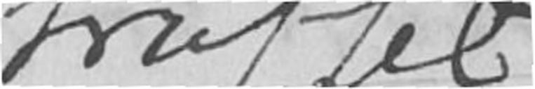truffet
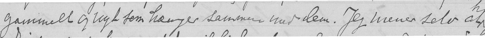gammelt og nyt som hænger sammen med dem. Jeg mener selv at
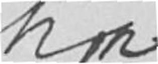ha
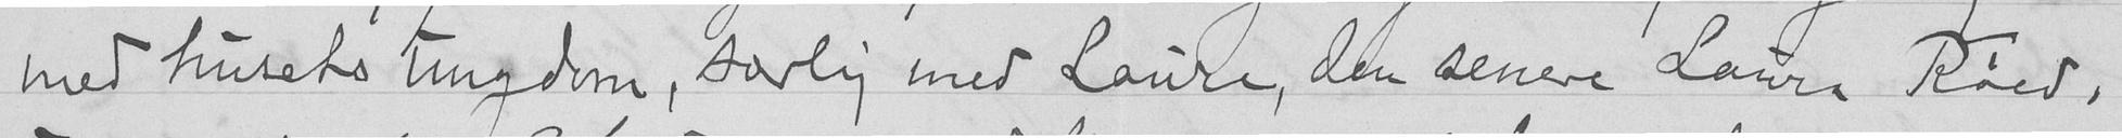med husets ungdom, særlig med Laura, den senere Laura Røed.
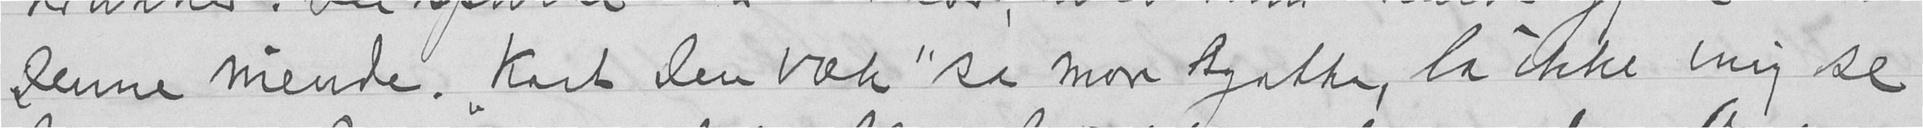denne niende. "Kast den væk" sa mor Agathe, la ikke mig se
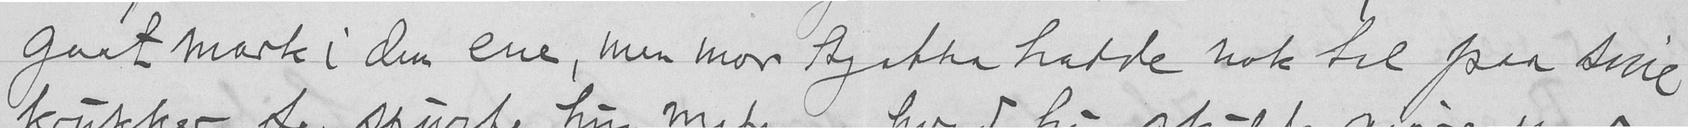gaat mark i den ene, men mor Agathe hadde nok til paa sine
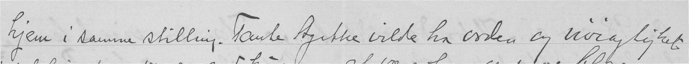hjem i samme stilling. Tante Agathe vilde ha orden og nøiagtighet
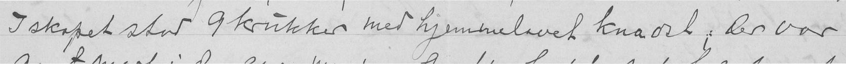I skapet stod 9 krukker med hjemmelavet knaost; der var
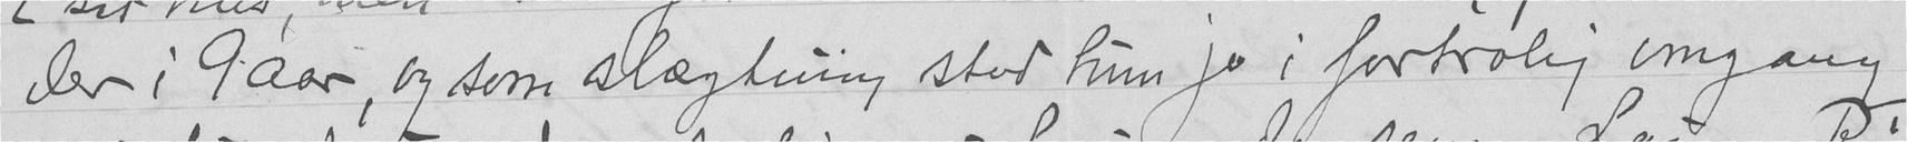der i 9 aar, og som slægtning stod hun jo i fortrolig omgang
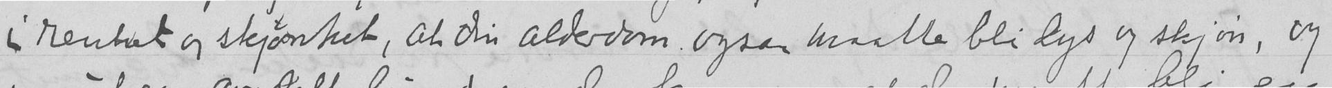i renhet og skjønhet, at din alderdom ogsaa maatte bli lys og skjøn, og
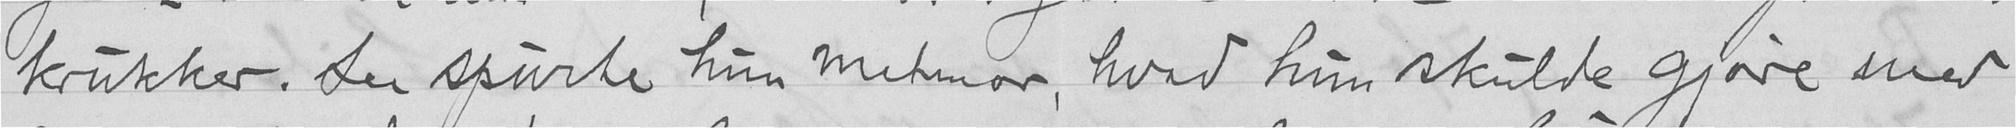krukker. Da spurde hun matmor, hvad hun skulde gjøre med
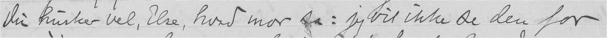"Du husker vel, Else, hvad mor sa: jeg vil ikke se den for
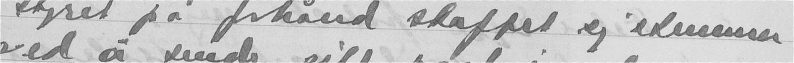styret på forhånd skaffet stemmer
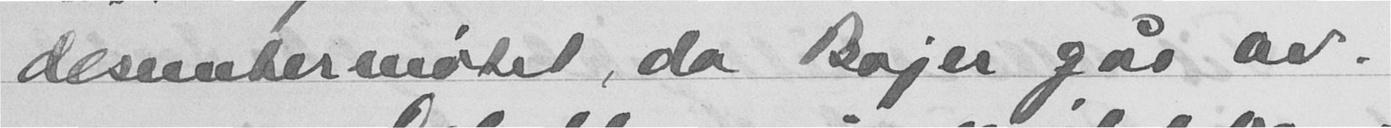desembermøtet, da Bojer går av.
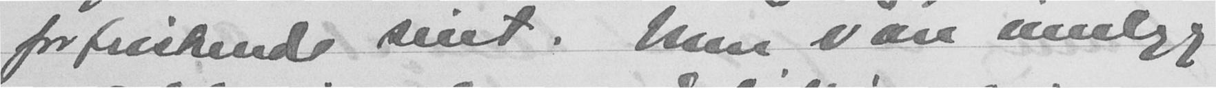forfriskende sint. Men våre innlegg
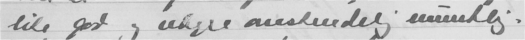lite god og uhyre omstendelig muntlig.
